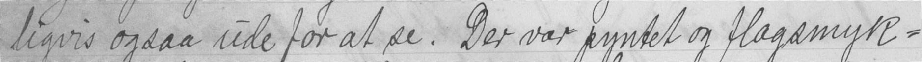ligvis ogsaa ude for at se. Der var pyntet og flagsmyk-
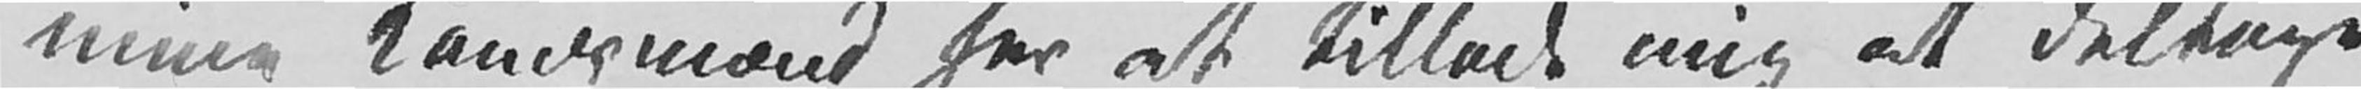mine Landsmænd her at tillade mig at deltage
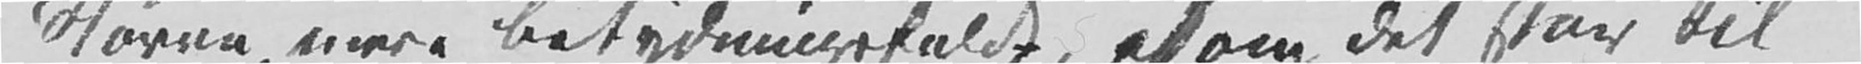Større, mere betydningsfuldt, som det står til
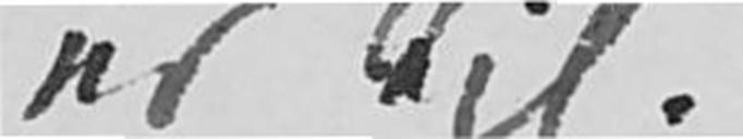er til.
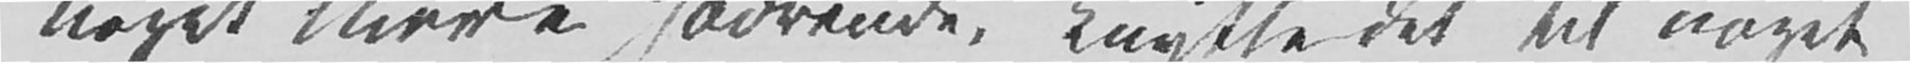noget mere hædrende, knytte det til noget
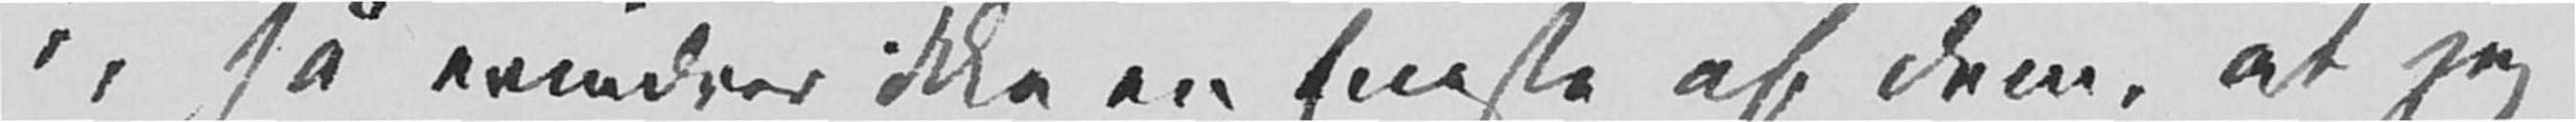i, så erindrer ikke en Eneste af dem, at jeg
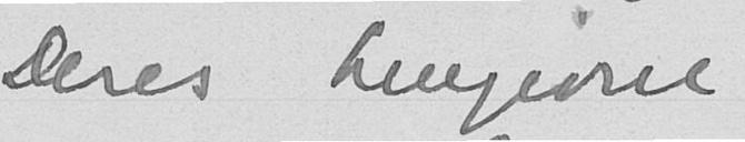Deres hengivne
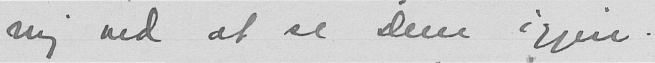mig ved at se Dem igjen.
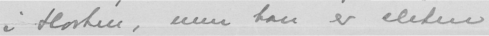i Horten, men han er sliten
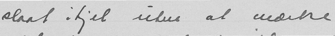slaat ihjel uten at mærke
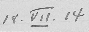18. VII. 14
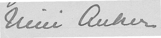Nini Anker
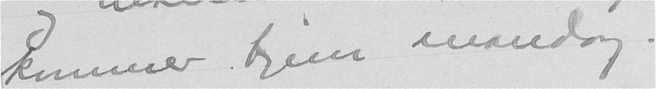kommer hjem mandag.
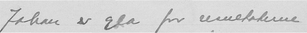Johan er gla for resultaterne
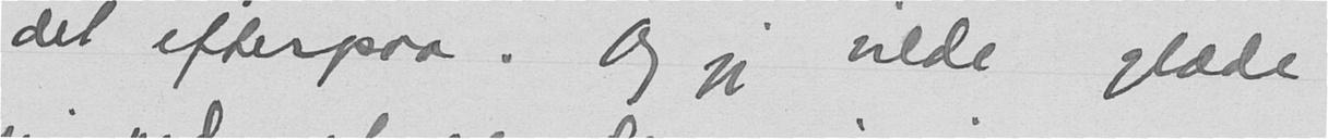det efterpaa. Og jeg vilde glæde
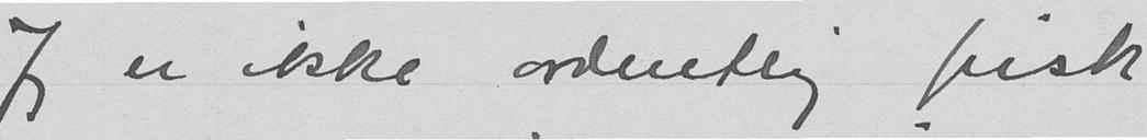Jeg er ikke ordentig frisk
